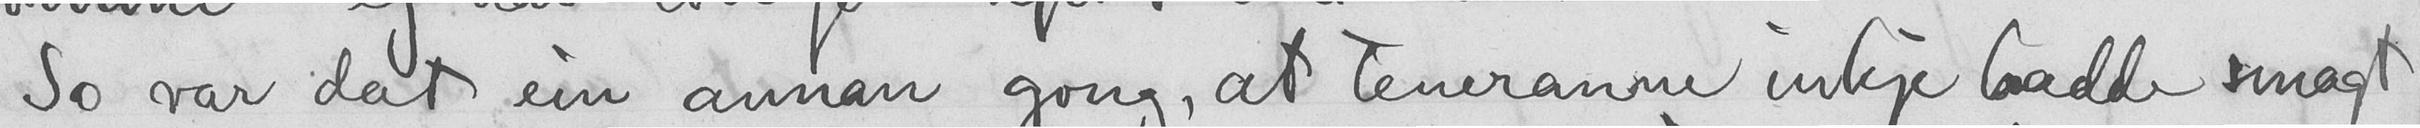sa var dat ein annan gong, at teneranne inkje hadde smagt
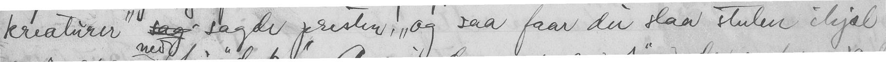kreaturer"  sagde presten, "og saa faar du slaa stuten ihjel
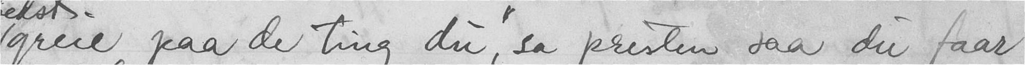greie paa de ting du," sa presten saa du faar
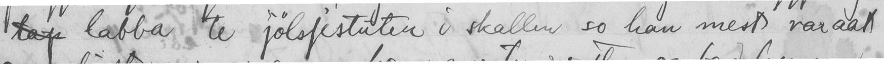labba te jølsjestuten i skallen so han mest var aat
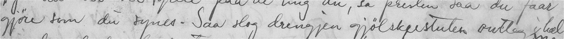gjøre som du synes. Saa slog drengjen gjølskjestuten ontleg ihæl
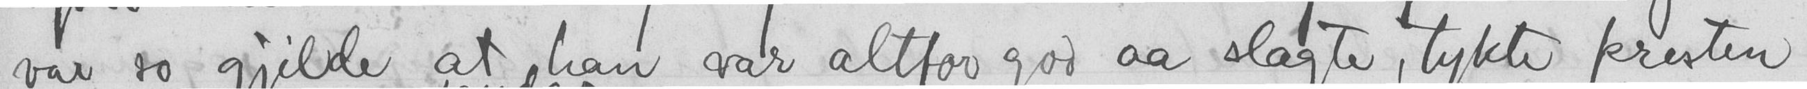var so gjilde at han var altfor god aa slagte, tykte presten
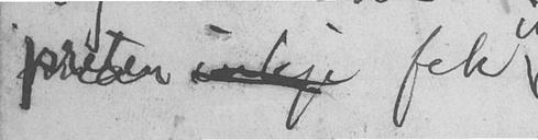presten  fek
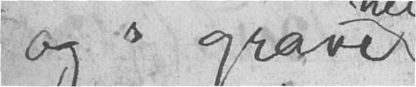og grave
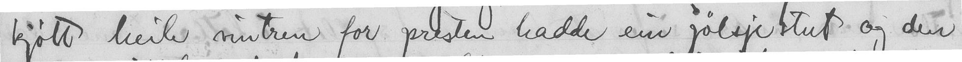kjøtt heile vintren for presten hadde ein jølsjestut og den
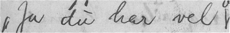"Ja du har vel
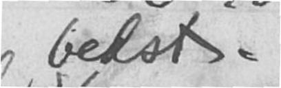bedst
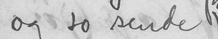og so sende
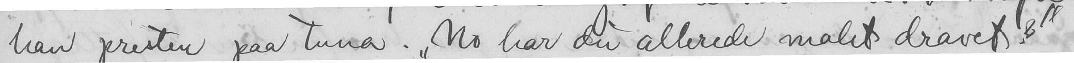han presten paa tuna. "No har du allerede malet dravet?"
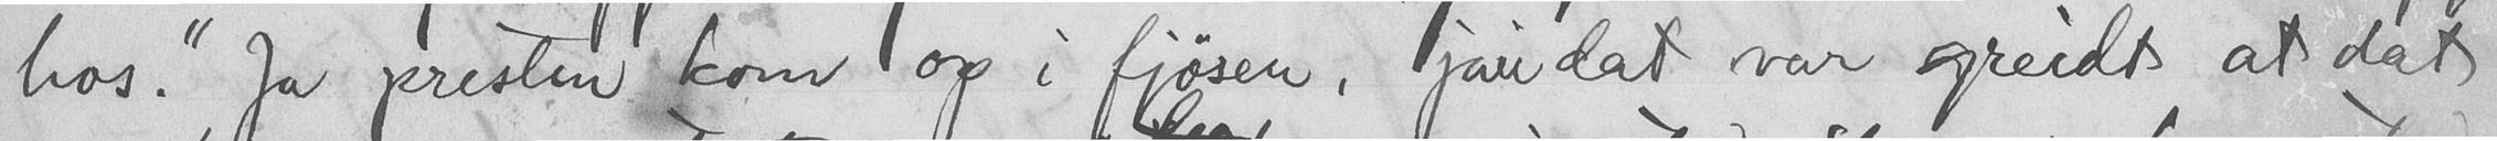hos." Ja presten kom op i fjøsen, jau dat var greidt at dat
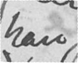han
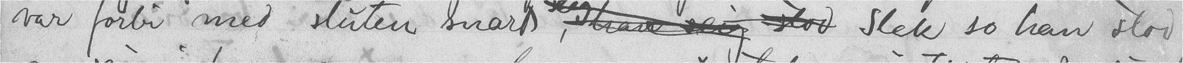var forbi med stuten snart  slek so han stod
